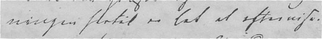ningen hertil er let at eftervise.
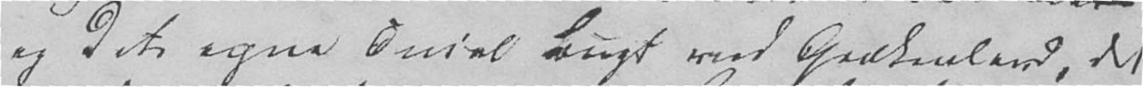og dets egne Tvivl Bugt med Grækenland, det
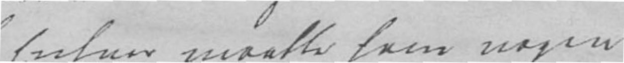Enhver maatte have nogen
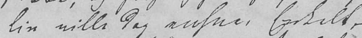Liv ville dog enhver Enkelt
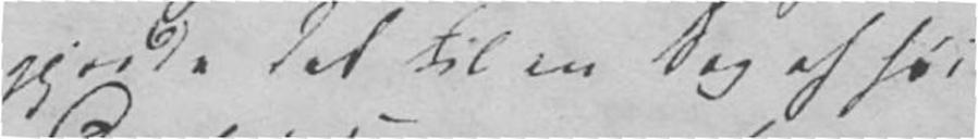gjorde det til en Sag af høi
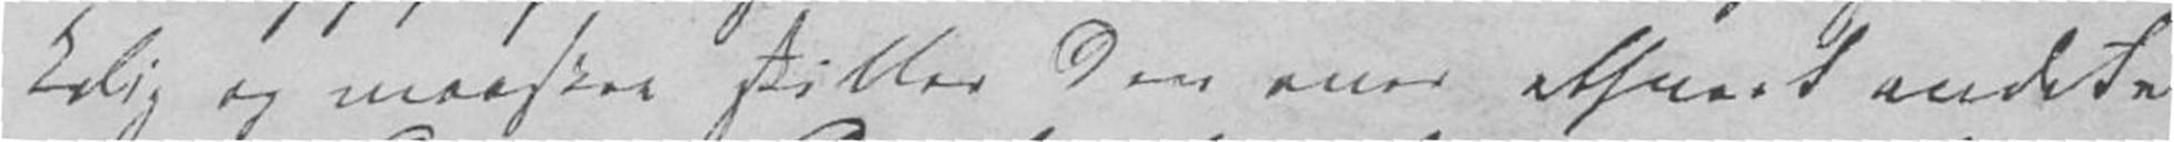kelig og maaskee stiller den over ethvert andet
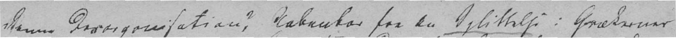denne Desorganisation? Aabenbar fra en Splittelse: Grækernes
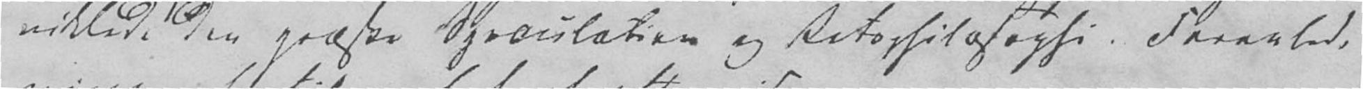viklede den græske Speculation og Retsphilosophi. Foranled-
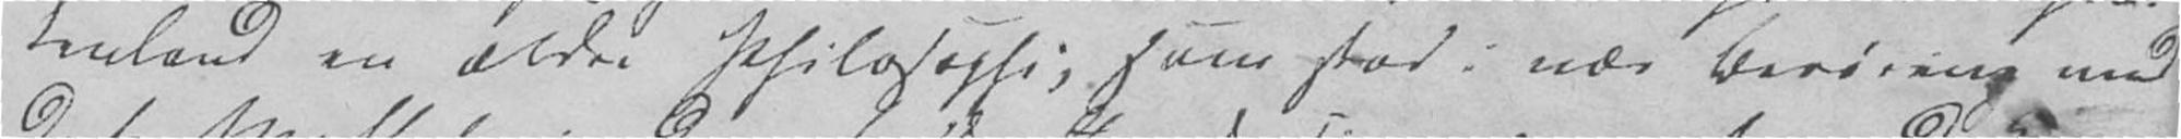kenland en ældre Philosophi, som stod i nær Berøring med
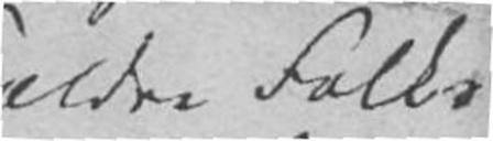ældre Folks.
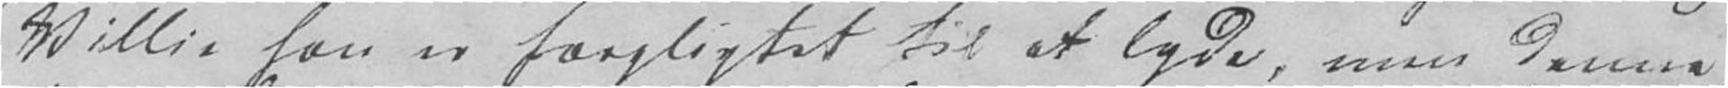Villie han er forpligtet til at lyde, men denne
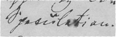Speculation.
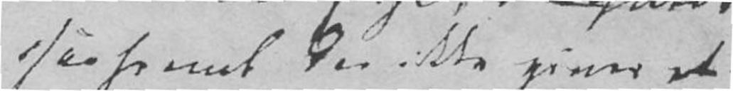saafremt der ikke gives et
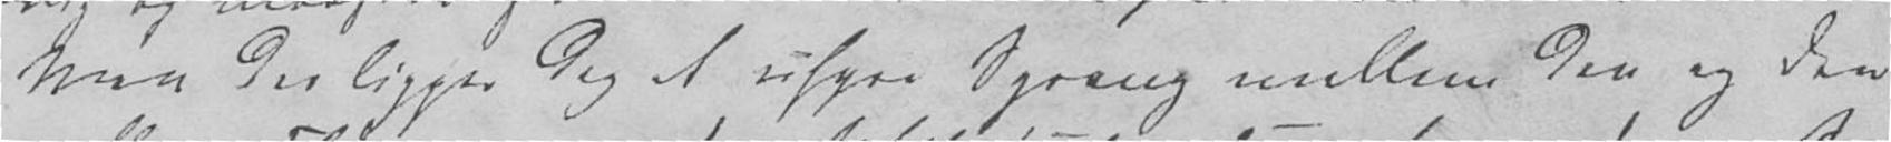Men der ligger dog et uhyre Sprang mellem den og den
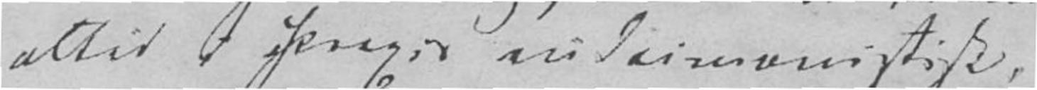altid i Praxis eudaimonistisk,
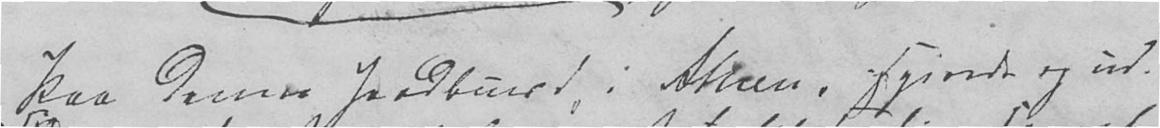Paa denne Jordbund i Athen, spirede og ud-
